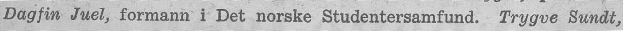Dagfin Juel, formann i Det norske Studentersamfund. Trygve Sundt,
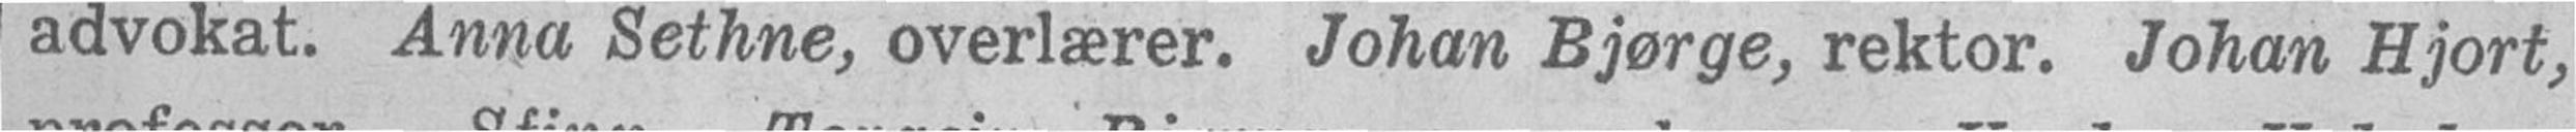advokat. Anna Sethne, overlærer. Johan Bjørge, rektor. Johan Hjort,
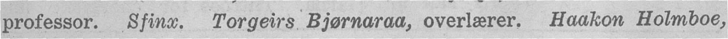professor. Sfinx. Torgeirs Bjørnaraa, overlærer. Haakon Holmboe,
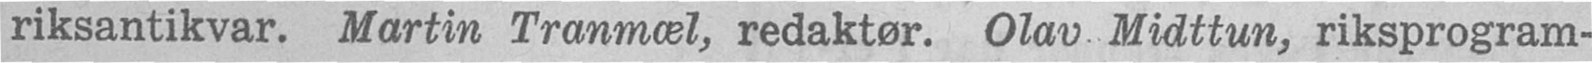riksantikvar. Martin Tranmæl, redaktør. Olav. Midttun, riksprogram-
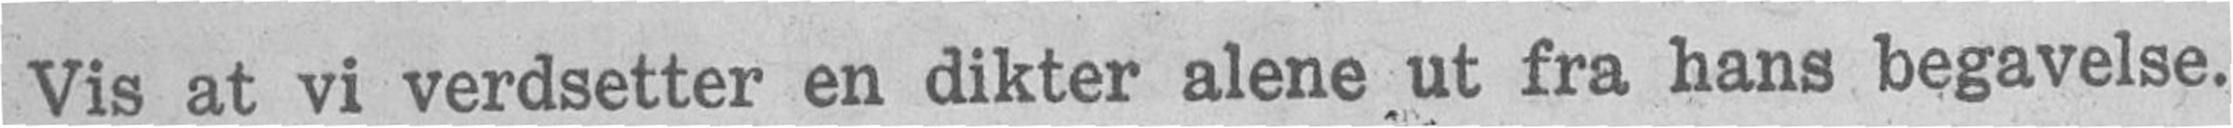Vis at vi verdsetter en dikter alene ut fra hans begavelse.
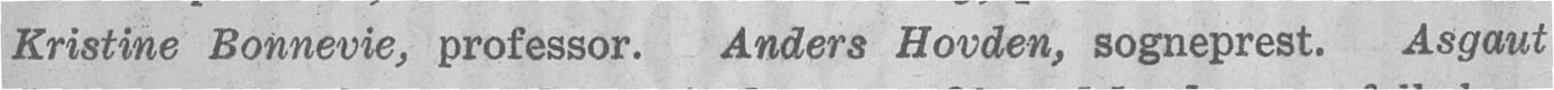Kristine Bonnevie, professor. Anders Hovden, sogneprest. Asgaut
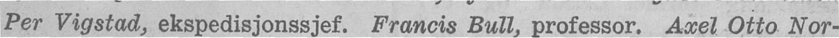Per Vigstad, ekspedisjonssjef. Francis Bull, professor. Axel Otto Nor-
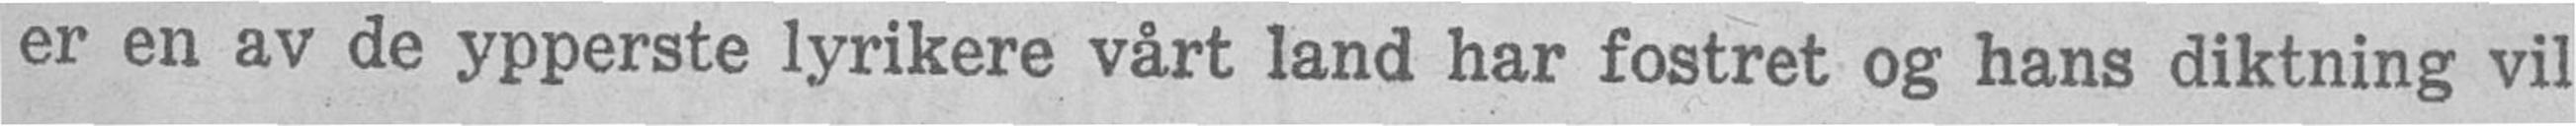er en av de ypperste lyrikere vårt land har fostret og hans diktning vil
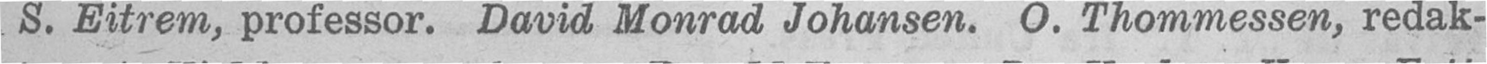S. Eitrem, professor. David Monrad Johansen. O. Thommessen, redak-
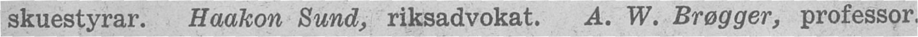skuestyrar. Haakon Sund, riksadvokat. A. W. Brogger, professor.
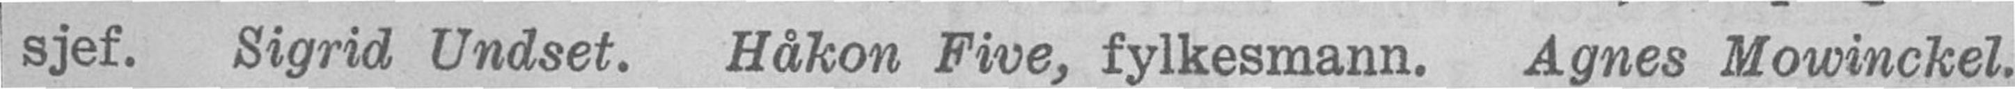sjef. Sigrid Undset. Håkon Five, fylkesmann. Agnes Mowinckel.
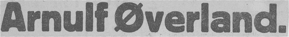Arnulf Øverland.
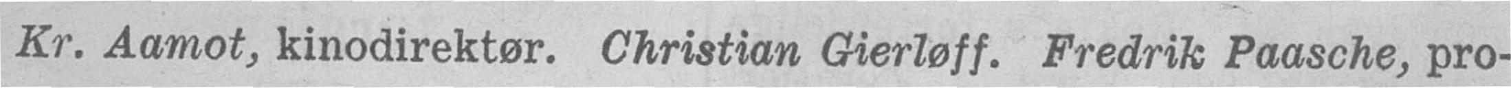Kr. Aamot, kinodirektør. Christian Gierløff. Fredrik Paasche, pro-
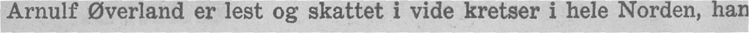Arnulf Øverland er lest og skattet i vide kretser i hele Norden, han
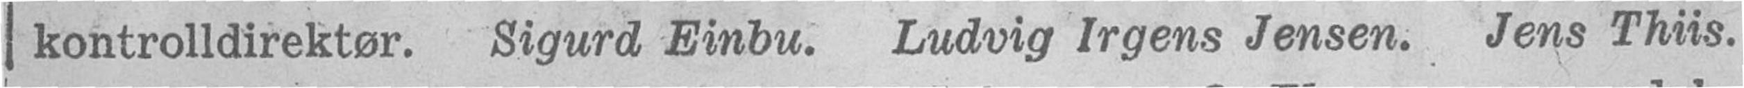kontrolldirektør. Sigurd Einbu. Ludvig Irgens Jensen. Jens Thiis.
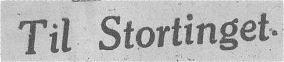Til Stortinget.
